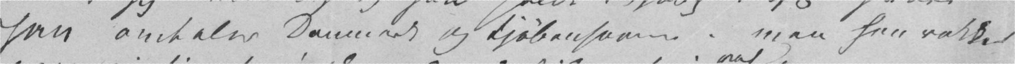han omtaler Danmark og Kjøbenhavnere, men han rakker
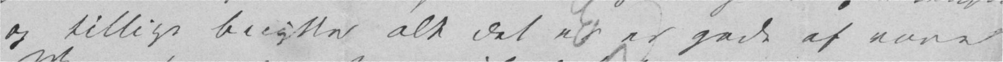og tillige benytte alt det er er godt af vore
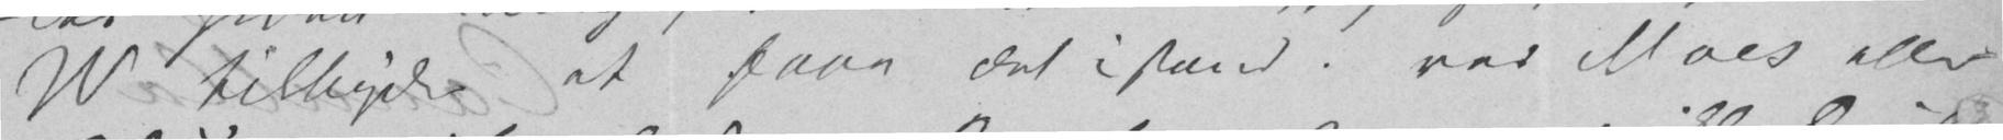W tilbyder at faae det istand. ved Moes eller
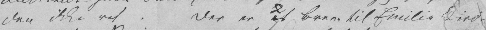den ikke ret. Der er et 2 Breve til Emilie Diriks
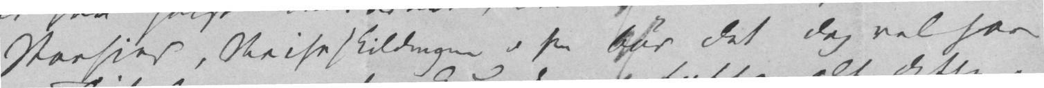Poesier, Reiseskildringer – saa bør det dog vel have
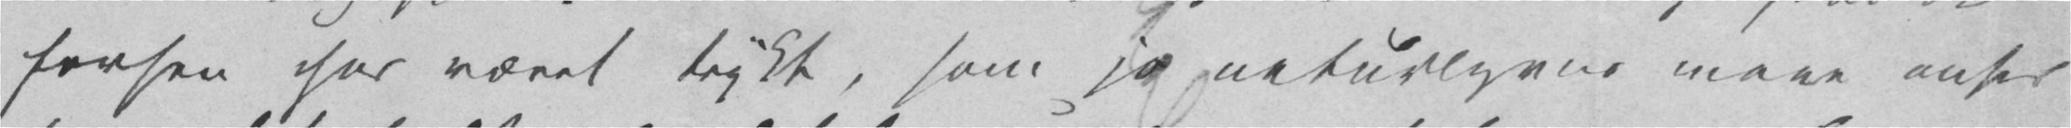forhen har været trykt, som jo naturligviis maae ansees
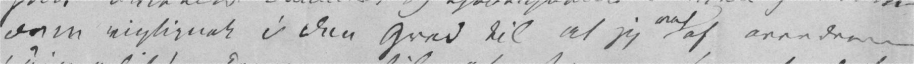dem rigtignok i den Grad til at jeg vel af overdreven
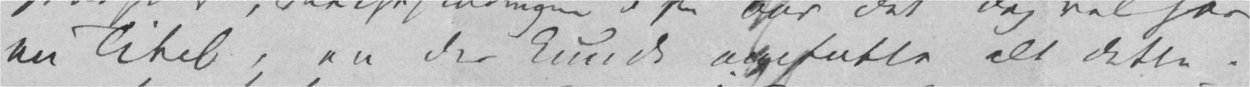en Titel, en der kunde omslutte alt dette.
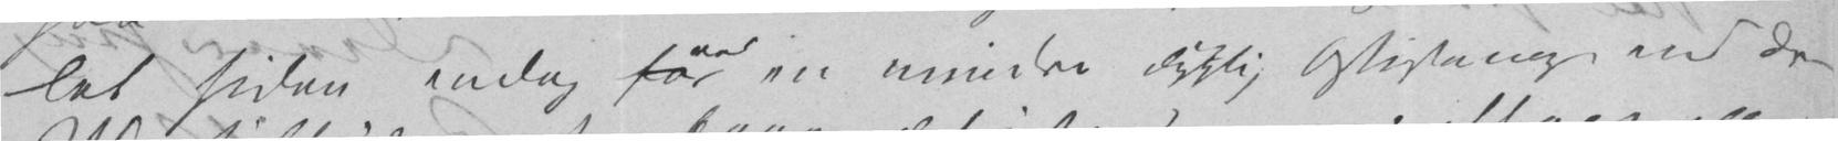let siden endog for ved en mindre dygtig Assistanze end den
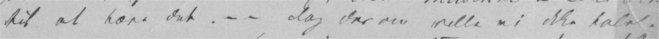til at bære det. – – Dog derom ville vi ikke tale.
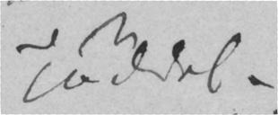Tøddel.
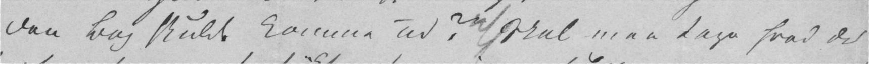den Bog skulde komme ud? Skal man tage hvad der
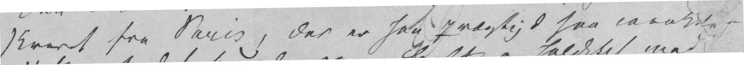skrevet fra Paris, der er saa prægtig & saa carakte-
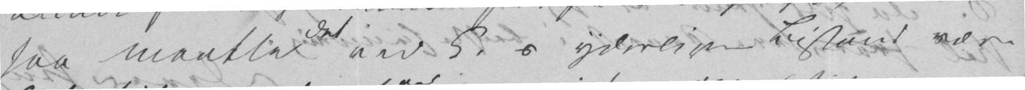saa maatte det ved C.s yderligere Bistand være
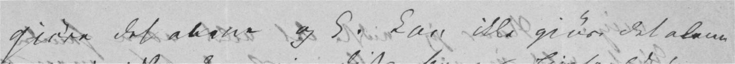gjøre det alene og C. kan ikke gjøre det alene
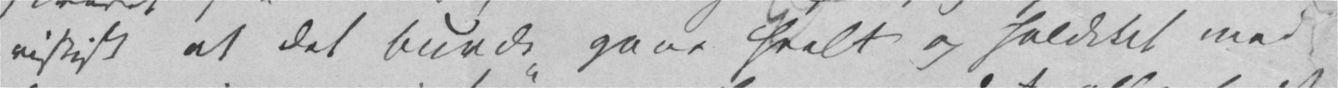ristisk at det burde gaae heelt og holdent med
